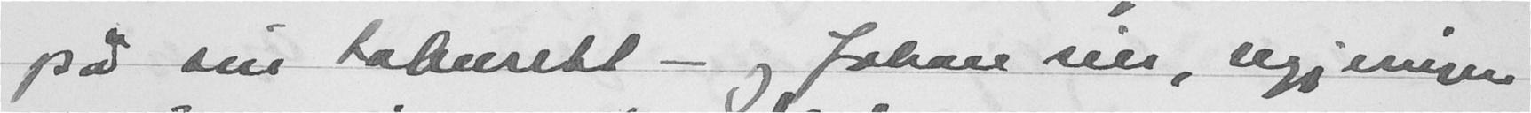på sin taburett - og Johan sier, regjeringen
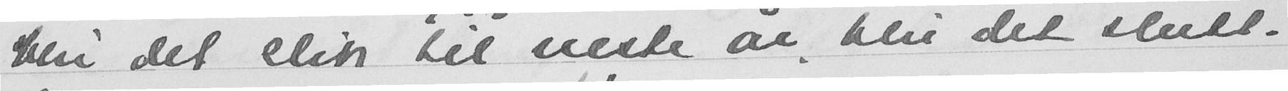blir det slik til neste år blir det slutt.
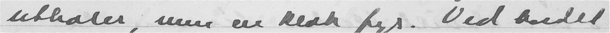uthaler, men en klok fyr. Ved bordet
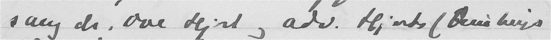sang dr. Ove Hjort og adv. Hjort (Quislings
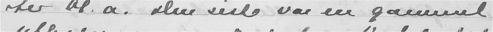ster bl. a. Han siste var en gammel
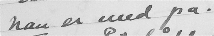han er med på.
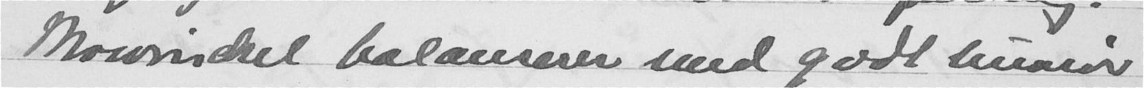Mowinckel balanserer med godt humør
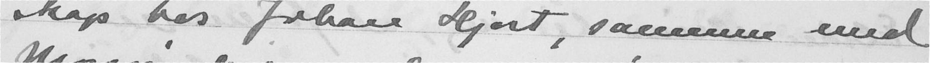skap hos Johan Hjort, sammen med
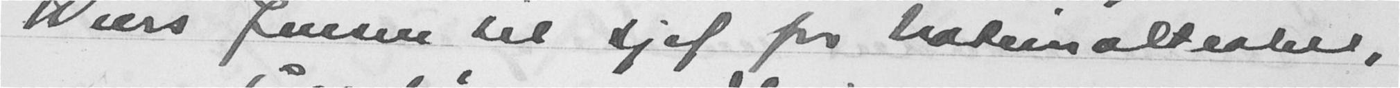Wiers Jensen til sjef for Nationaltheateret,
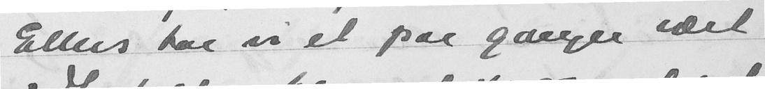Ellers har vi et par ganger vært
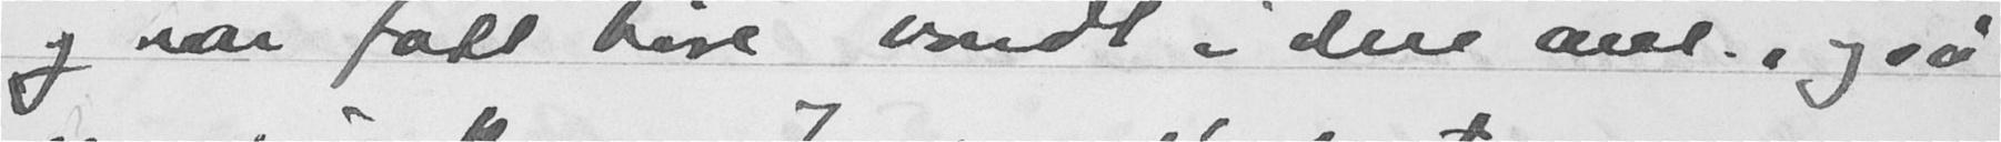og har fått høre vondt i den anl, også
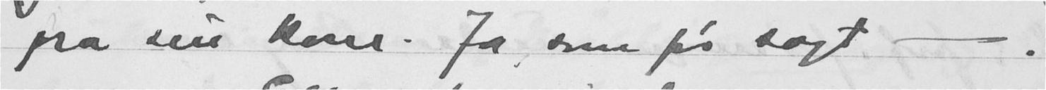fra sin kone. Ja, som før sagt - .
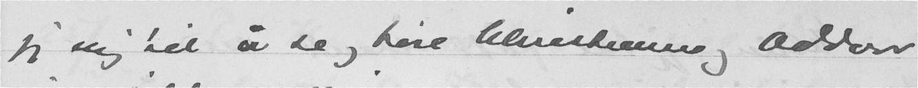jeg mig til å se og høre Christensen og Oddvar
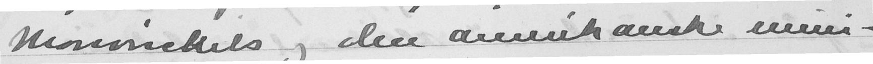Mowinckel og den amerikanske minis-
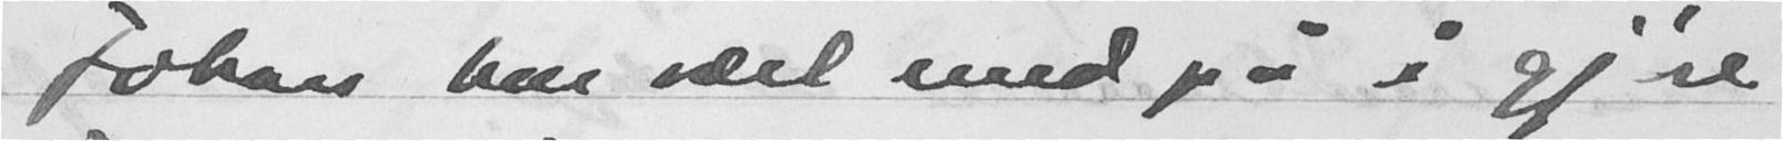Johan her vært med på å gjøre
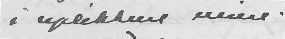i replikkene mine.
